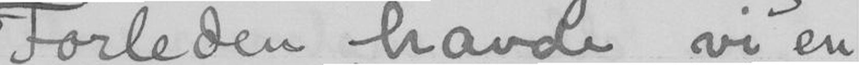Forleden havde vi en
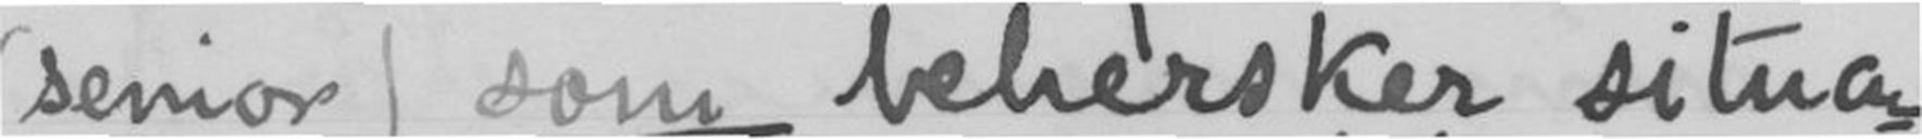senior) som behersker situa
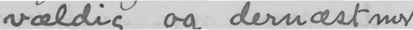vældig og dernæst mod
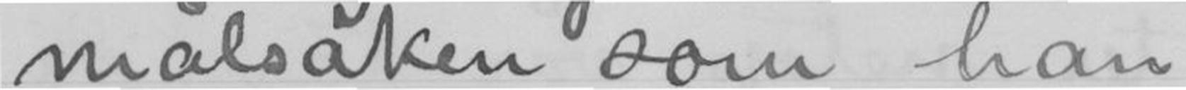målsaken som han
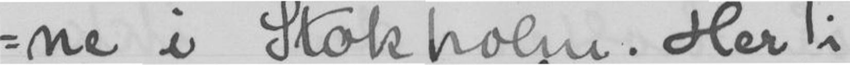ne i Stokholm. Her i
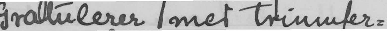Gratulerer med triumfer-
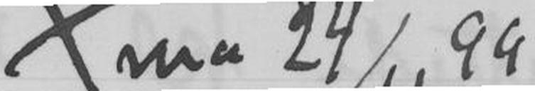Xnia 24/11 99
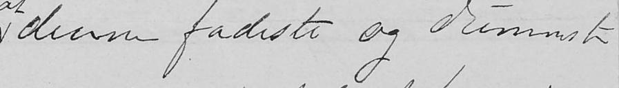denne fæleste og dummeste
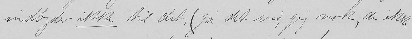indbyder ikke til det, (ja det ved, jeg nok, de ikke
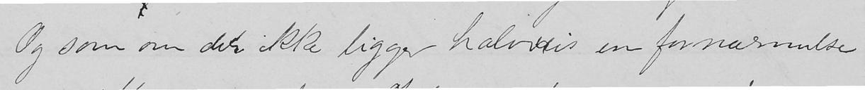Og som om der ikke ligger halvveis en fornærmelse
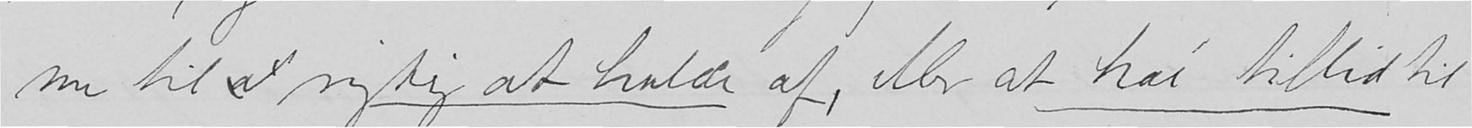me til at rigtig at holde af, eller at hae' tillid til
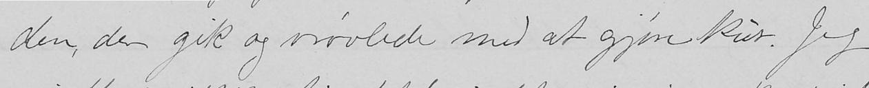den, der gik og vrøvlede med at gjøre kur. Jeg
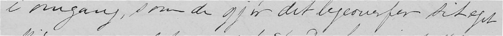i omgang, som de gjør det ligeoverfor sit eget
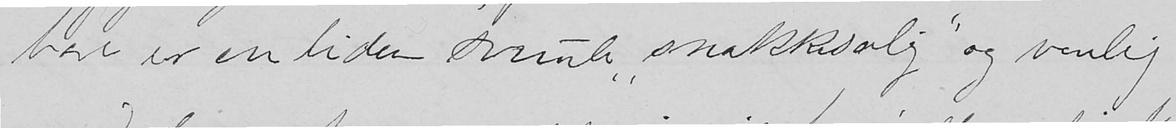bare er en liden smule, "snakksalig" og venlig
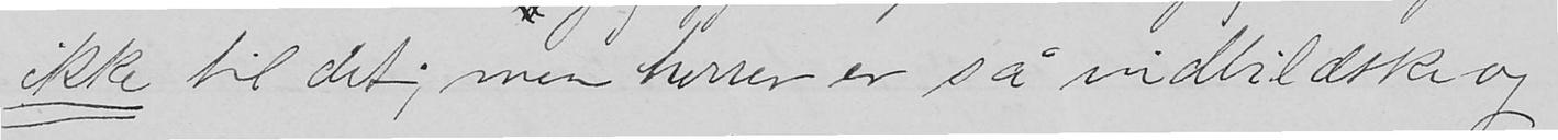ikke til det; men herrer er så indbildske og
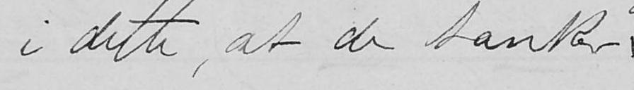i dette, at de tænker
